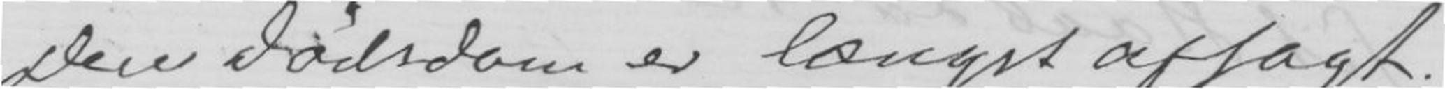Den dødsdom er længst afsagt.
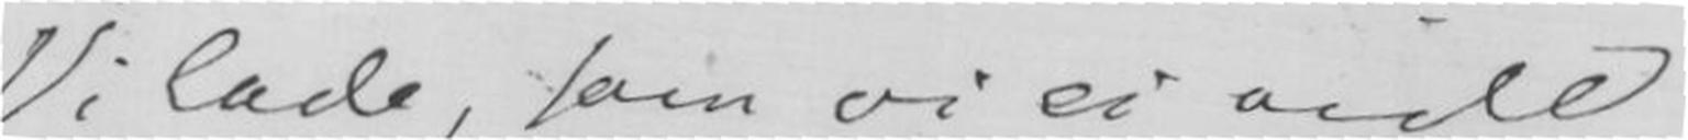Vi lade, som vi ei vide
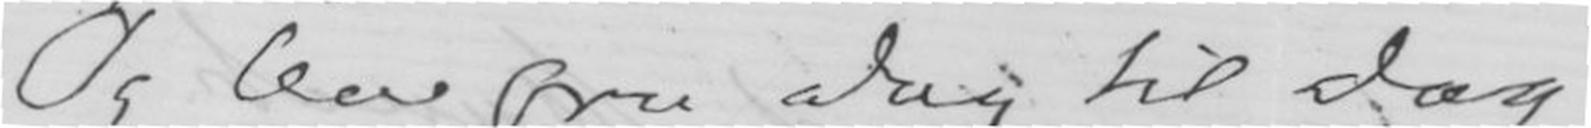Og leve fra dag til dag
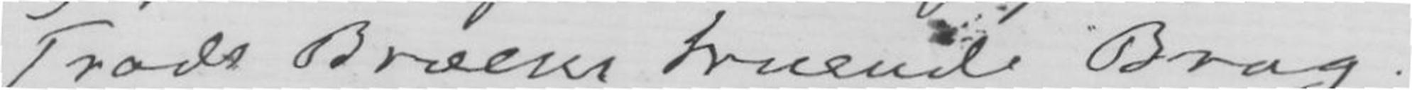Trods Bræens truende Brag.
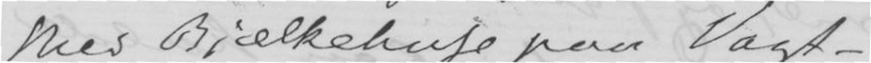sker Bjælkehuse paa Vagt -
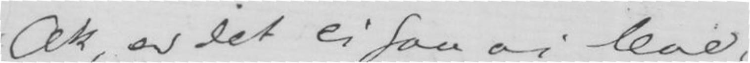Ak, er det ei saa vi leve,
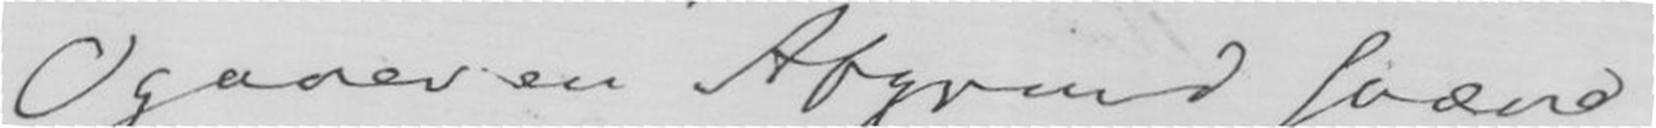Og over en Afgrund svæve
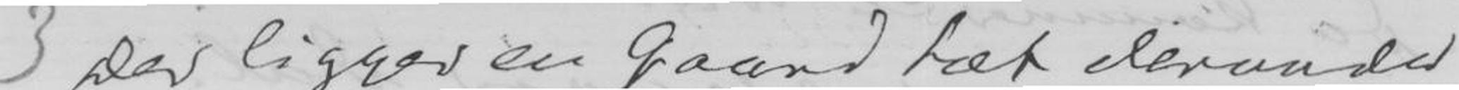3 Der ligger en Gaard tæt derunder
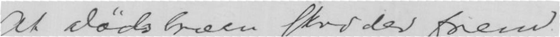At dødsbræen skrider frem
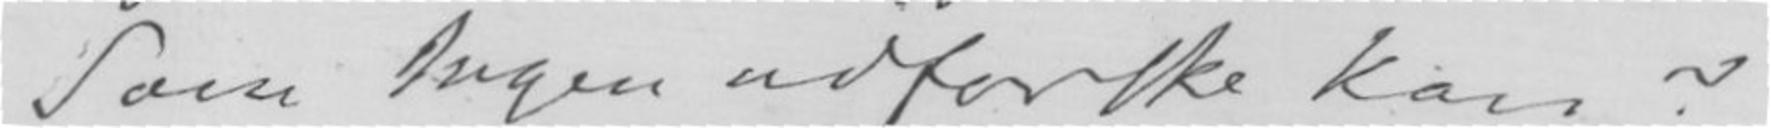Som Ingen udforske kan?
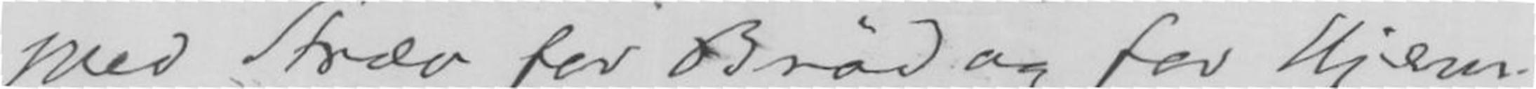Med Stræv for Brød og for Hjem.
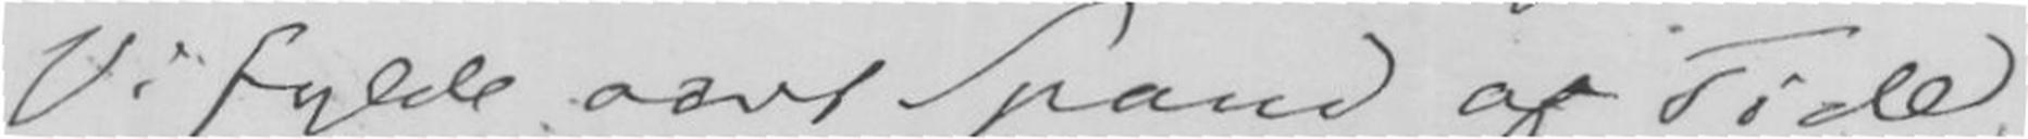Vi fylde vort Spand af Tide.
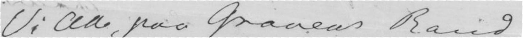Vi Alle, paa Gravens Rand
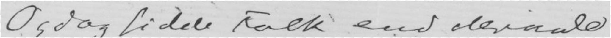Og dog sidde Folk end derunder
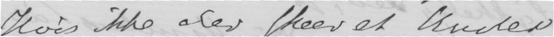Hvis ikke der skeer et Under
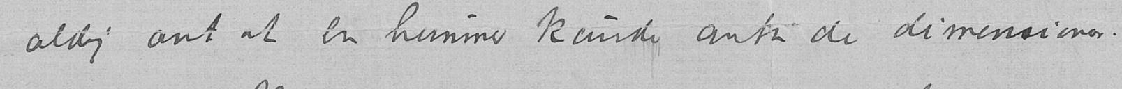aldrig ant at en hummer kunde anta de dimensioner.
